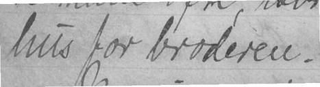hus for broderen.
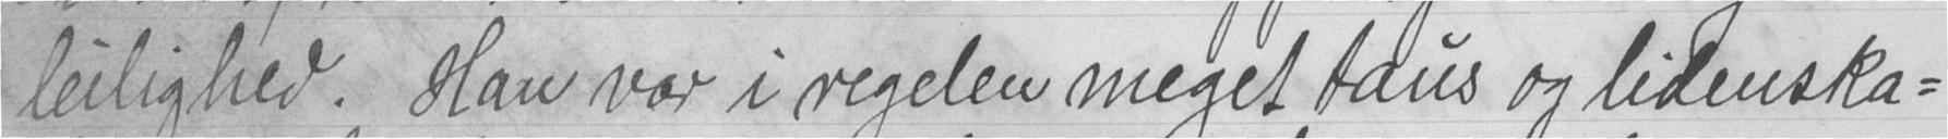leilighed. Han var i regelen meget taus og lidenska-
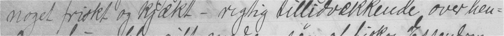noget friskt og kjækt - rigtig tillidvækkende over hen-
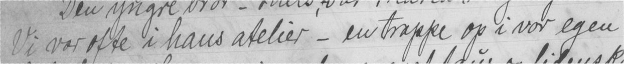Vi var ofte i hans atelier - en trappe op i vor egen
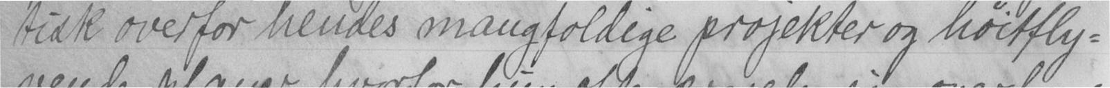tisk overfor hendes mangfoldige prosjekter og høitfly-
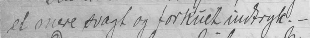et mere svagt og forkuet indtryk. -
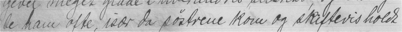te ham ofte, især da søstrene kom og skiftevis holdt
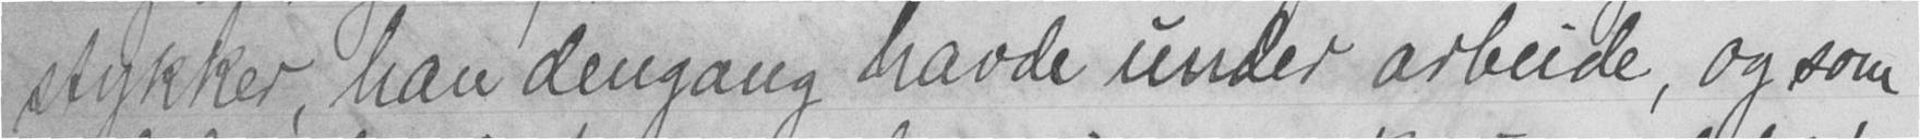stykker, han dengang havde under arbeide, og som
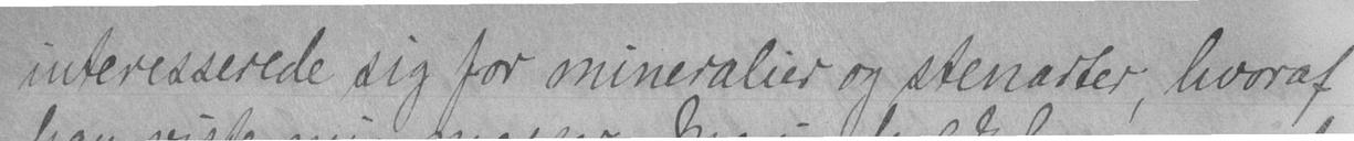interesserede sig for mineralier og stenarter, hvoraf
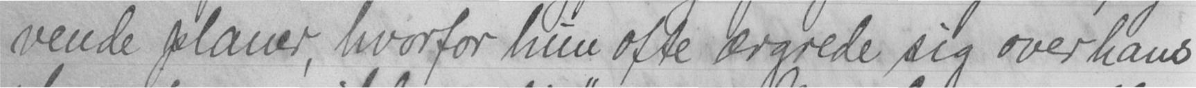vende planer, hvorfor hun ofte ærgrede sig over hans
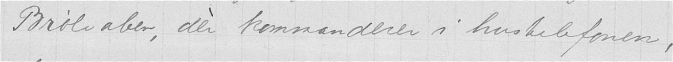Brøleaben, der kommanderer i hustelefonen,
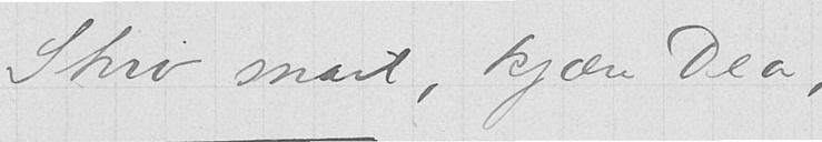Skriv snart, kjære Dea,
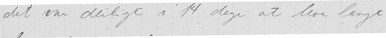det var deiligt i 14 dage at leve langt
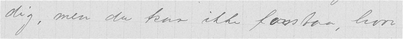dig, men du kan ikke forstaa, hvor
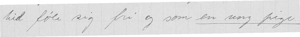tid føle sig fri og som en ung pige.
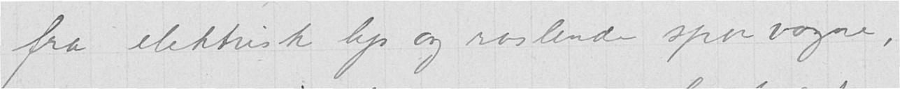fra elektrisk lys og raslende sporvogne,
